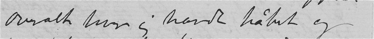overalt hvor jeg havde håbet og
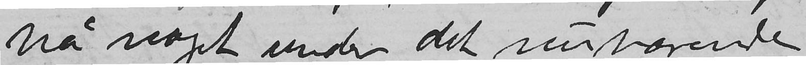nå noget under det nuværende
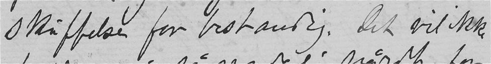skuffelse for bestandig. Det vel ikke
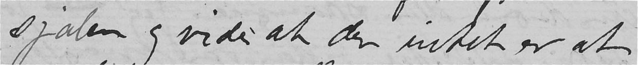sjælen og vide at der intet er at
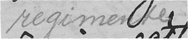regimente
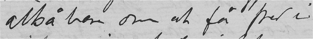altså bare om at få fred i
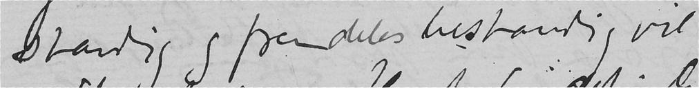standig og fremdeles bestandig vil
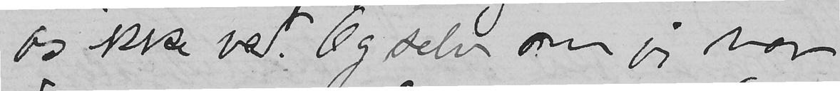os ikke ved. Og selv om jeg var
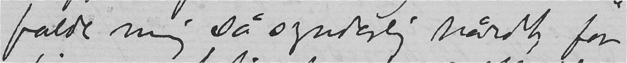falde mig så synderlig hårdt for
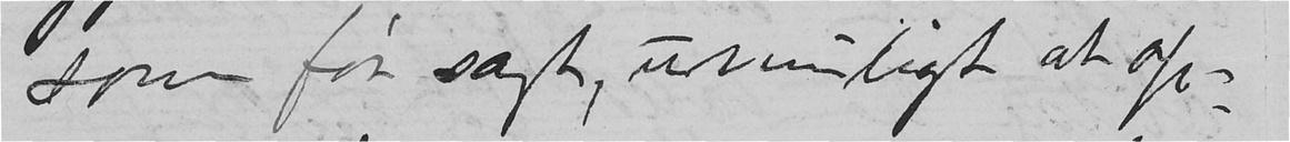som før sagt, umuligt at op-
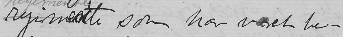regimette som har været be-
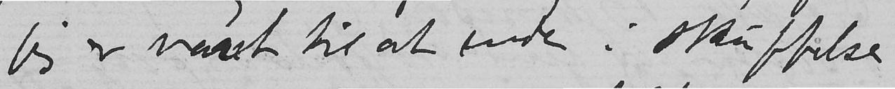jeg er vant til at ende i skuffelse
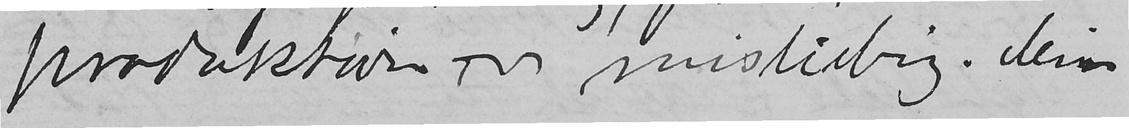produktion misliebig. Min
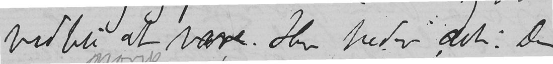vedbli at være. Her heder det: De
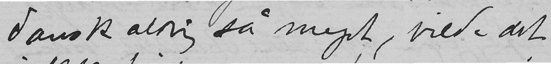dansk aldrig så meget, vilde det
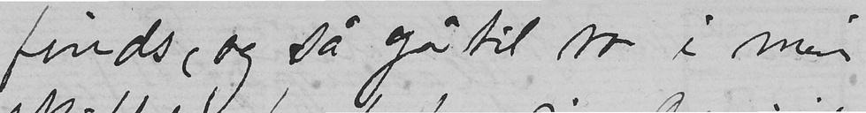finds, og så gå til ro i min
